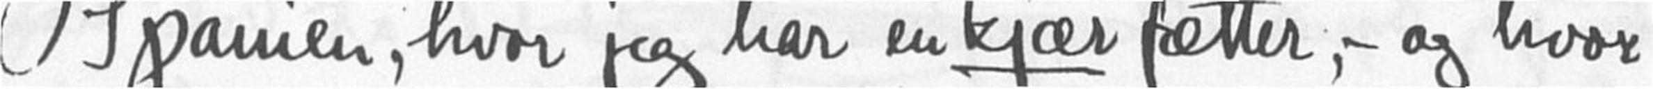Spanien, hvor jeg har en kjær fætter, - og hvor
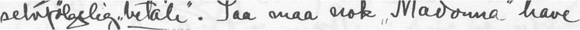selvfølgelig "betale". Saa maa nok "Madonna" have
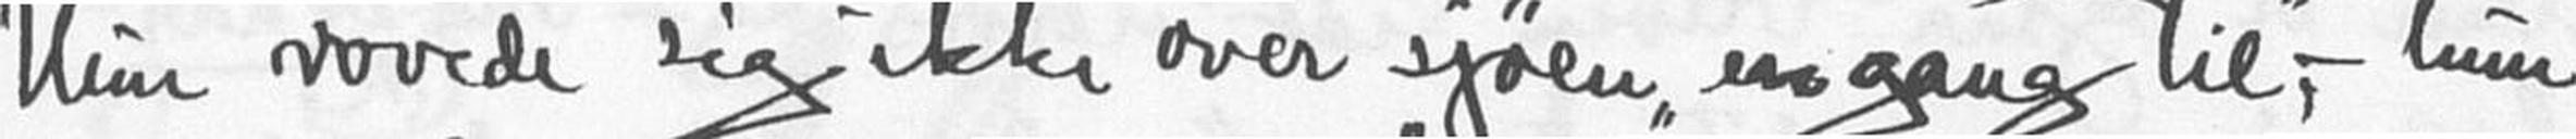Hun vovede sig ikke over sjøen "engang til", - hun
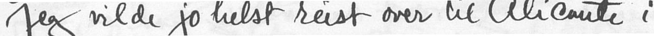Jeg vilde jo helst reist over til Alicante i
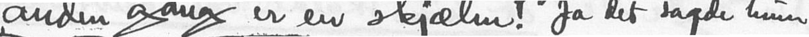anden gang er en skjælm !" Ja det sagde hun
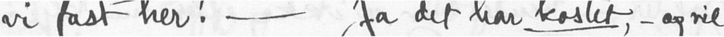vi fast her ! - Ja det har kostet, - og vil
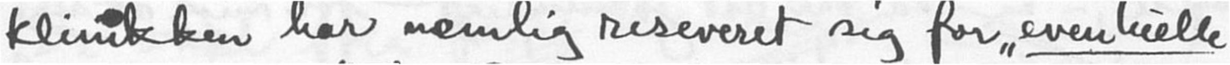klinikken har nemlig reserveret sig for "eventuelle
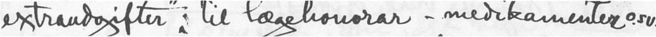extraudgifter"; til lægehonorar - medikamenter o.s.v.
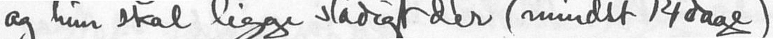og hun skal ligge stadigt der (mindst 14 dage).-
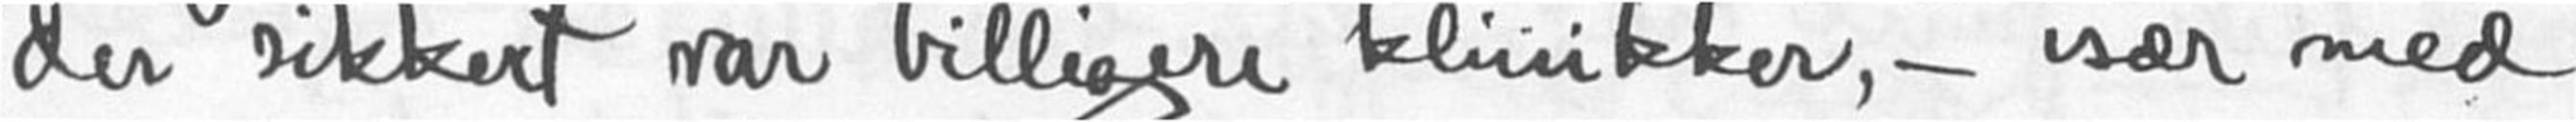der sikkert var billigere klinikker, - især med
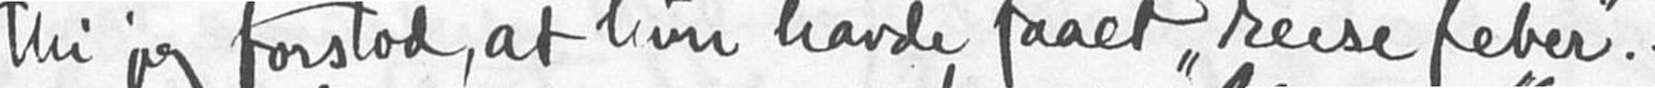thi jeg forstod, at hun havde faaet "reisefeber". -
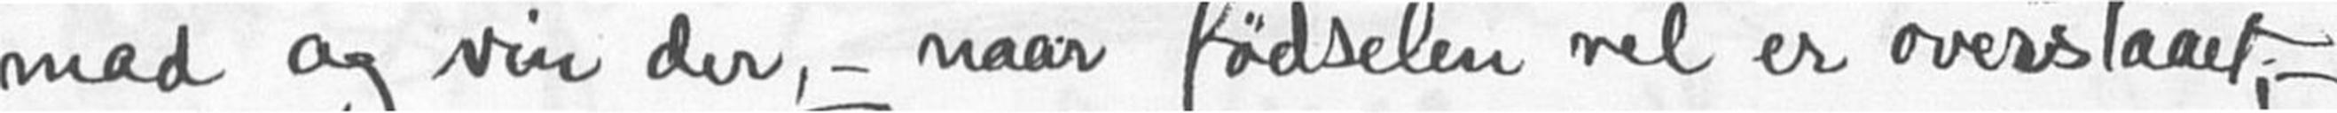mad og vin der, - naar fødselen vel er overstaaet;-
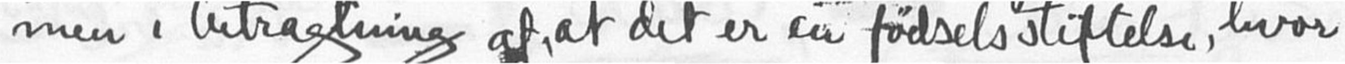men i betragtning af, at det er en fødselsstiftelse, hvor
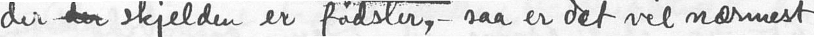der skjelden er fødsler, - saa er det vel nærmest
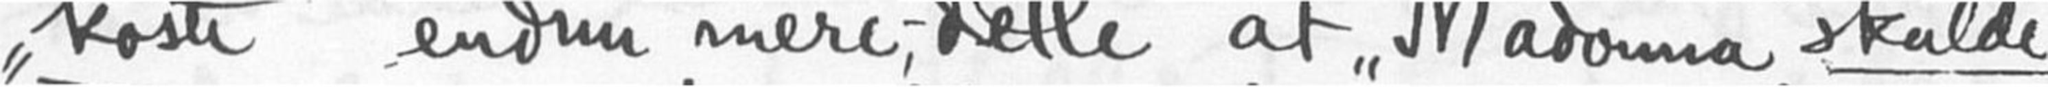"koste" endnu mere, dette at "Madonna" skulde
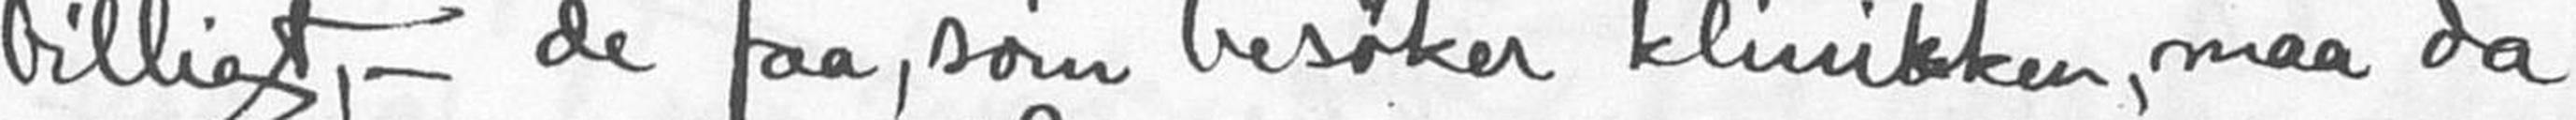billigt, - de faa, som besøker klinikken, maa da
